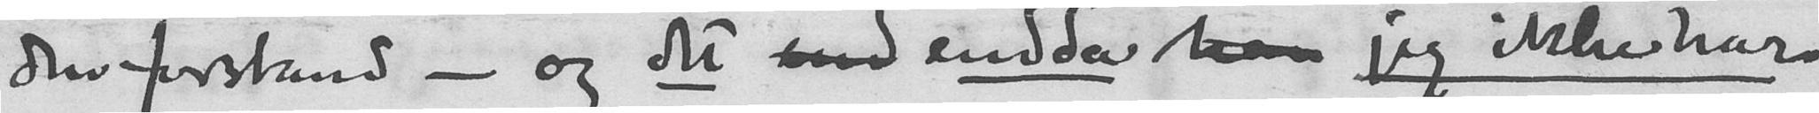den forstand - og det end endda han jeg ikke har
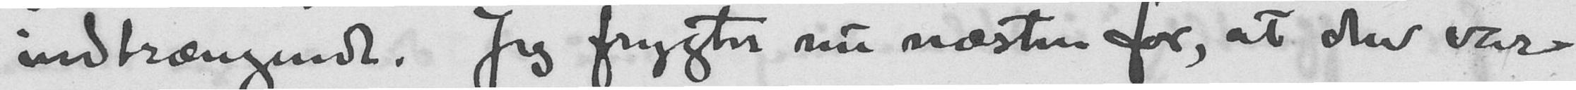indtrængende. Jeg frygter nu næsten for, at den var
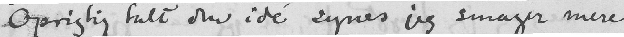Oprigtig talt den idé synes jeg smager mere
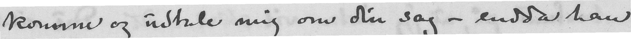komme og udtale mig om din sag - endda han
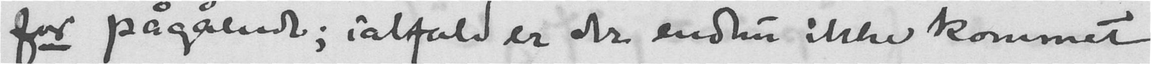for pågående; ialfald er der endnu ikke kommet
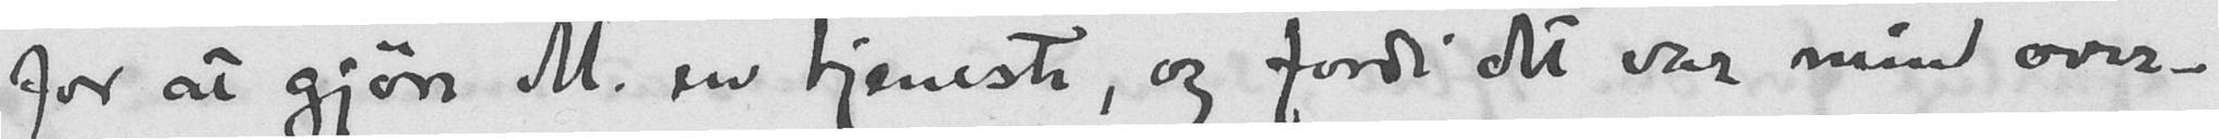for at gjøre M. en tjeneste, og fordi det var min over-
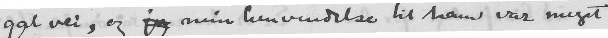gal vei, og jeg min henvendelse til ham var meget
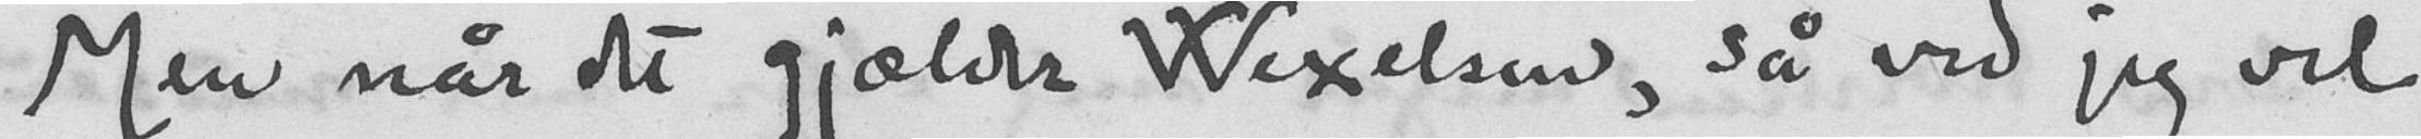Men når det gjælder Wexelsen, så ved jeg vel
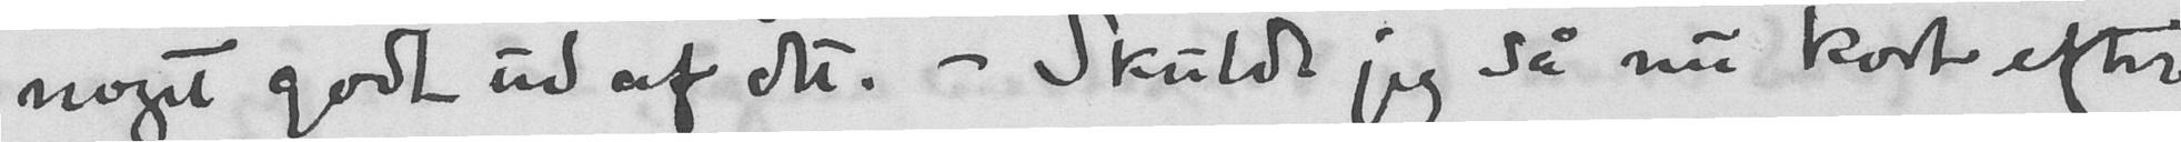noget godt ud af det. - Skulde jeg så nu kort efter
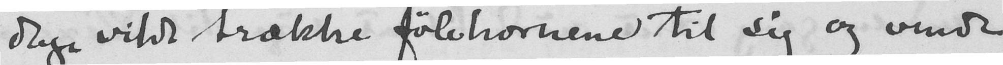dage vilde trække følehornene til sig og vende
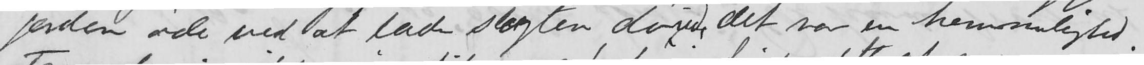jorden øde ved at lade slægten dø ut, det var en hemmelighed.
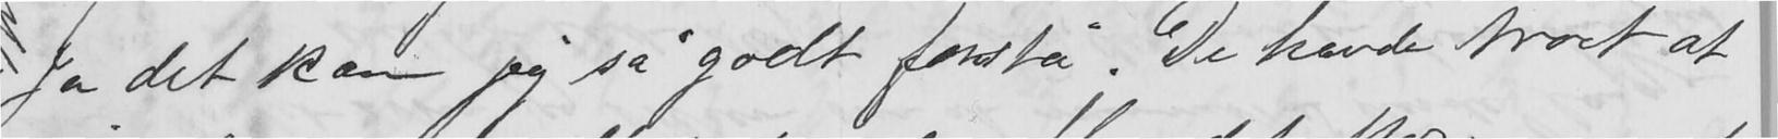Ja det kan jeg s godt forstå. De havde troet at
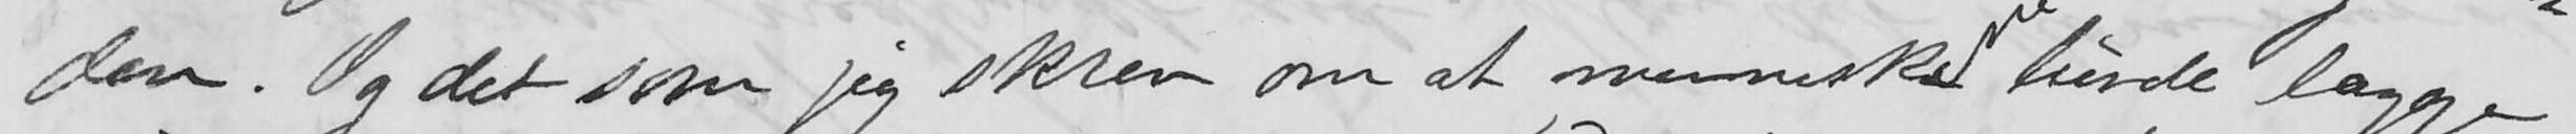den. Og det som jeg skrev om at menneskene burde lægge
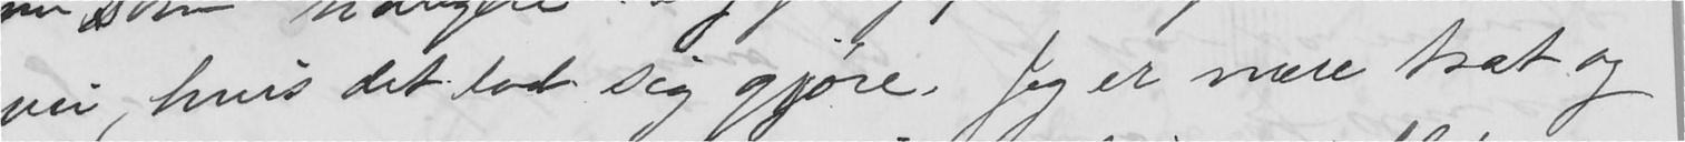vei, hvis det lot sig gjøre. Jeg er mere træt og
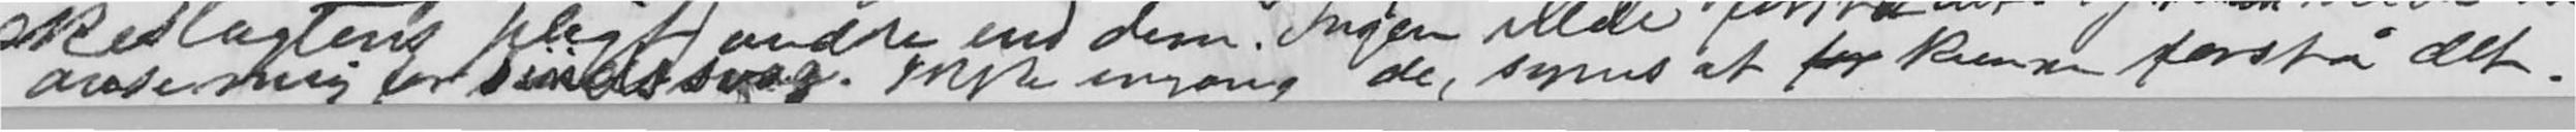anse mig for sindsvag. Ikke engang de, synes at kunne forstå det.
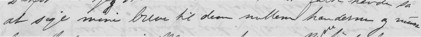at sige mine breve til dem mellem hænderne og mund-
